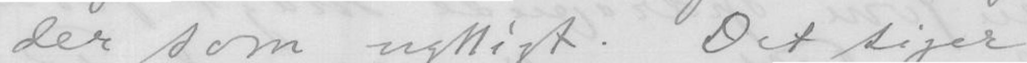der som nyttigt. Det siger
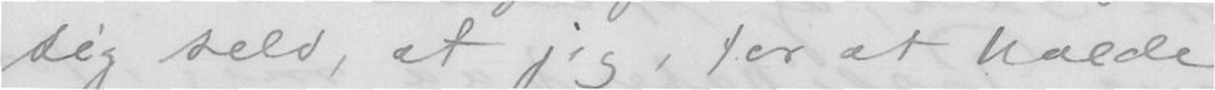sig selv, at jeg, for at halde
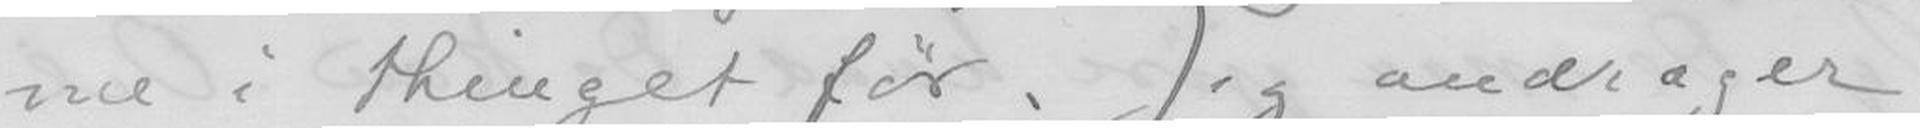me i thinget før. Jeg andrager
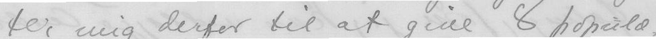ter mig derfor til at give 8 populæ-
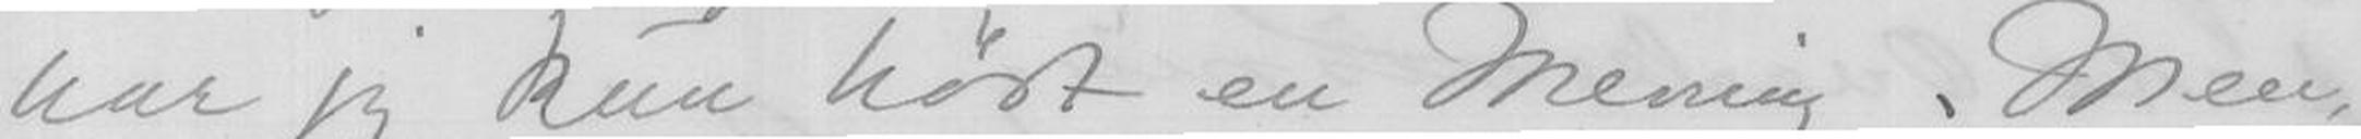har jeg kun hørt en Mening. Men
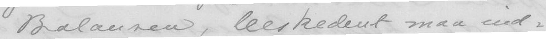Balansen, beskedent maa ind-
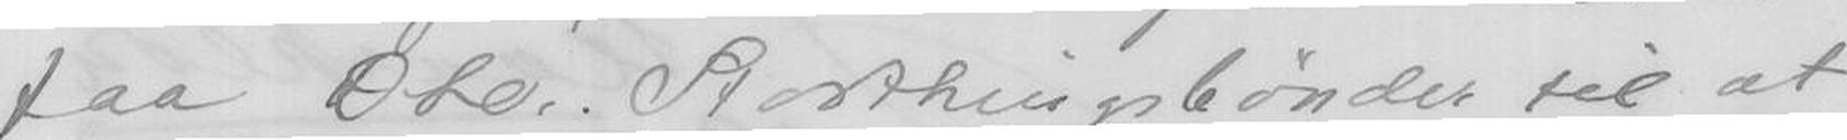faa alle Storthingsbønder til at
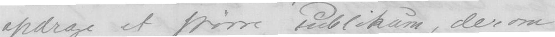opdrage at prøve Publikum, derom
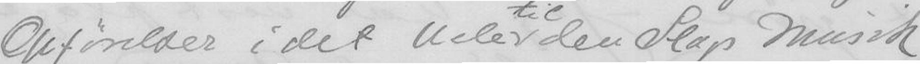Opførelser i det Hele den Slags Musik,
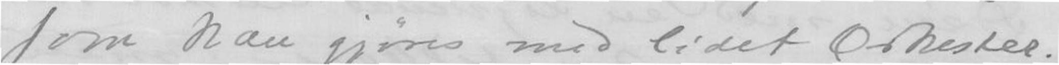som kan gjøres med lidet Orkester.
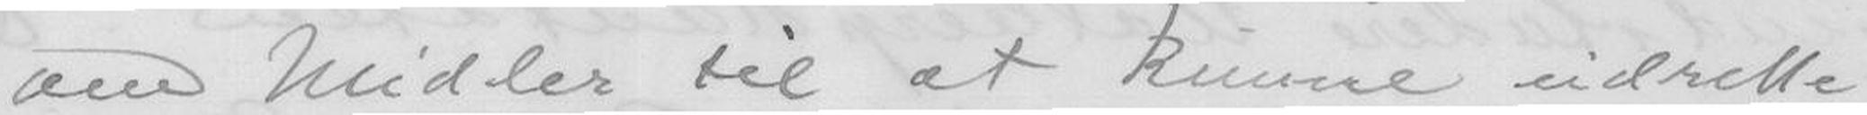om Midler til at kunne udrette
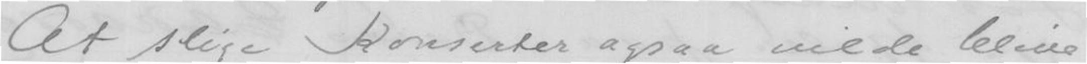At slige Konserter ogsaa vilde blive
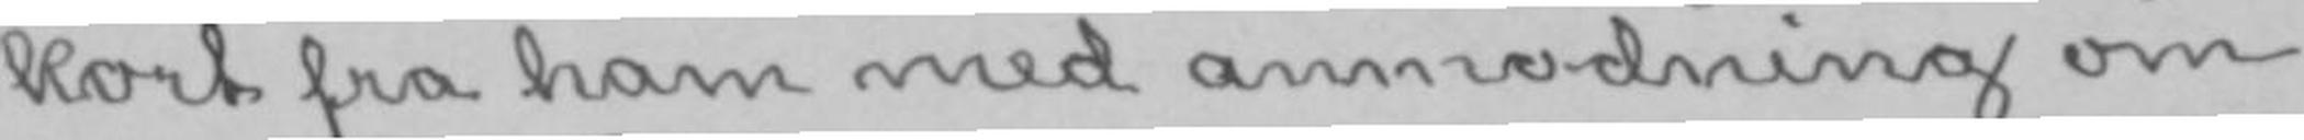kort fra ham med anmodning om
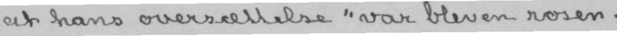at hans oversættelse «var bleven rosen-
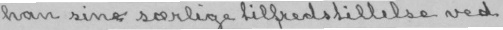han sine særlige tilfredstillelse ved
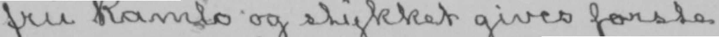fru Ramlo og stykket gives første
